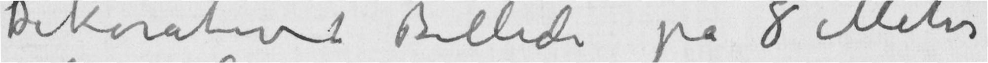Dekorativt Billede på 8 Meter
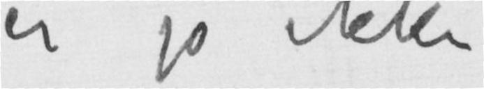er jo ikke
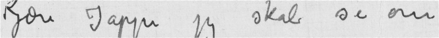Kjære Jappe jeg skal se om
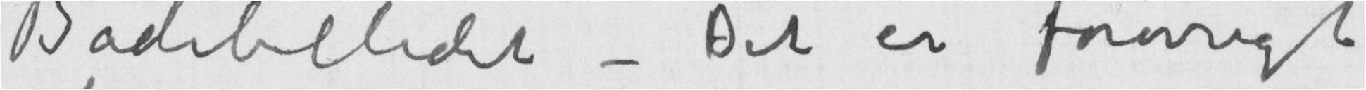Badebilledet – Det er forøvrigt
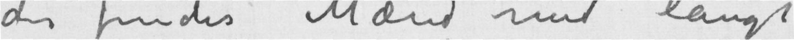der findes Mænd med langt
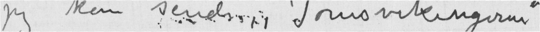jeg kan sende «Jomsvikingerne»
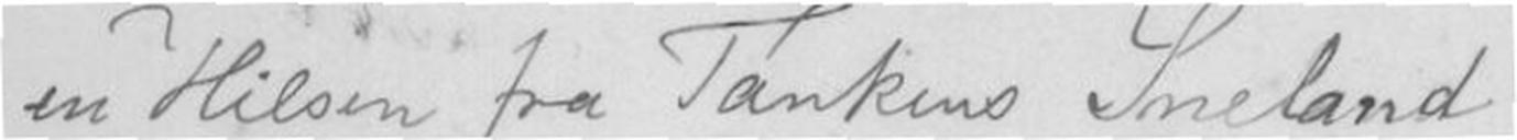en Hilsen fra Tankens Sneland
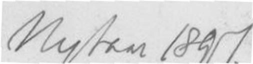Nytaar 1897.
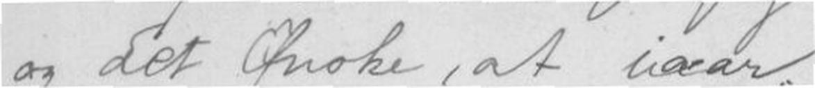og det Ønske, at iaar,
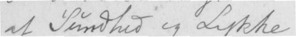af Sundhed og Lykke
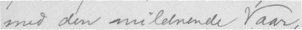med den mildnende Vaar,
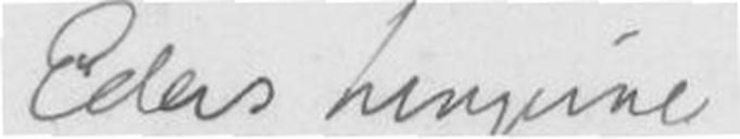Eders hengivne
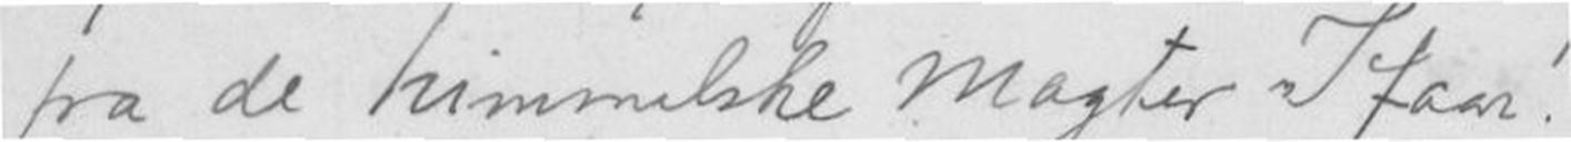fra de himmelske Magter Ifaar!
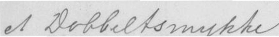et Dobbeltsmykke
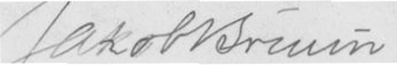Jakob Bruun
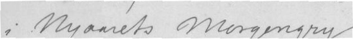i Nyaarets Morgengry,
